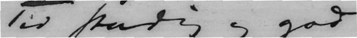Tid stadig og god!
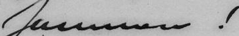sammen!
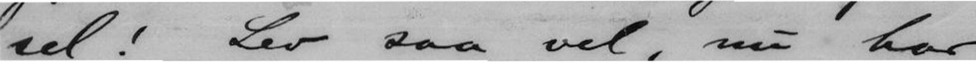sel! Lev saa vel, nu har
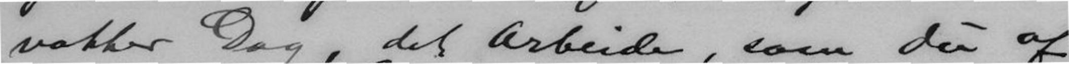vakker Dag, det Arbeide, som du af
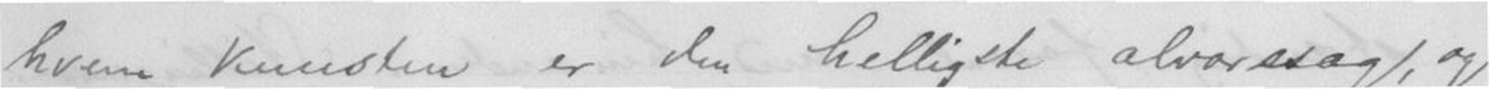hvem kunsten er den helligste alvorssag, og
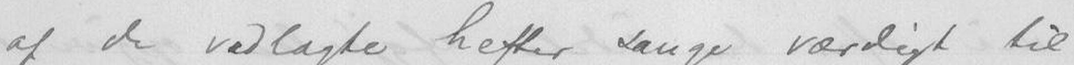af de vedlagte hefter sange værdigt til
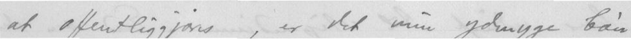at offentliggjøre, er det min ydmyge bøn,
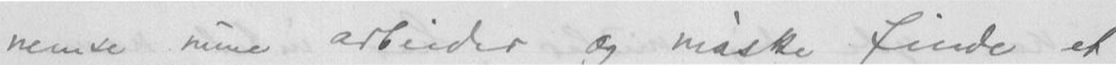nemse mine arbeider og måske finde et
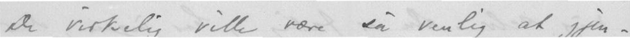De virkelig ville være så venlig at gjen-
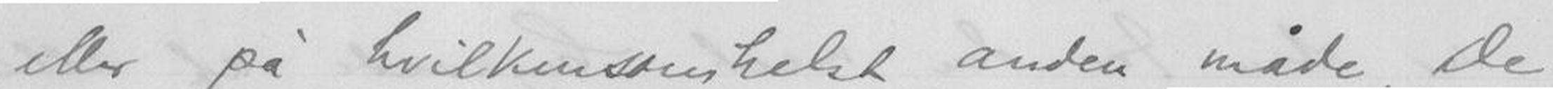eller på hvilkensomhelst anden måde, De
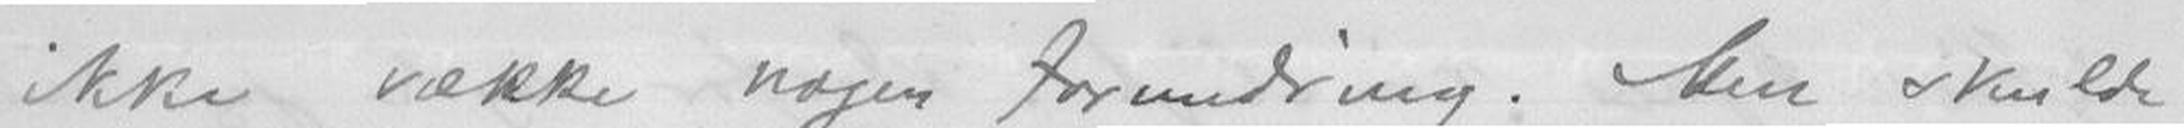ikke vække nogen forandring. Men skulde
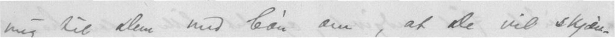mig til Dem med bøn om, at De vil skjænke
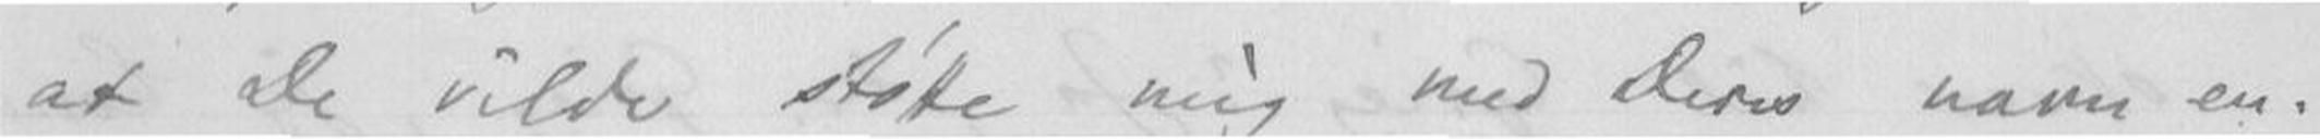at De vilde støte mig med Deres navn en-
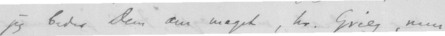jeg beder Dem om meget, hr. Grieg, men
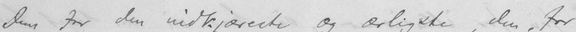Dem for den nidkjæreste og ærligste, den for
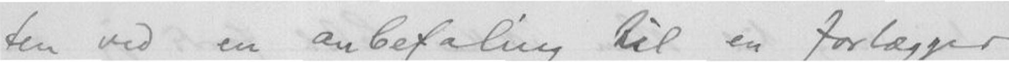ten ved en anbefaling til en forlægger
